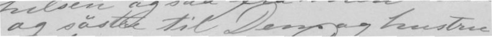og søster til Dem og hustru
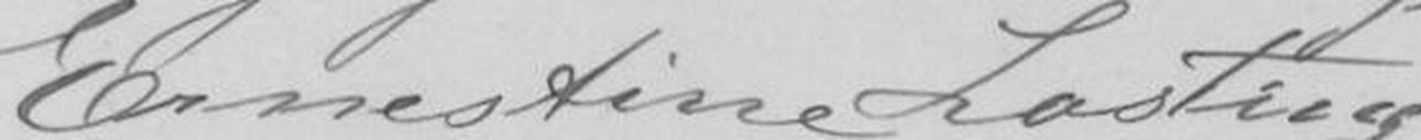Ernestine Losting.
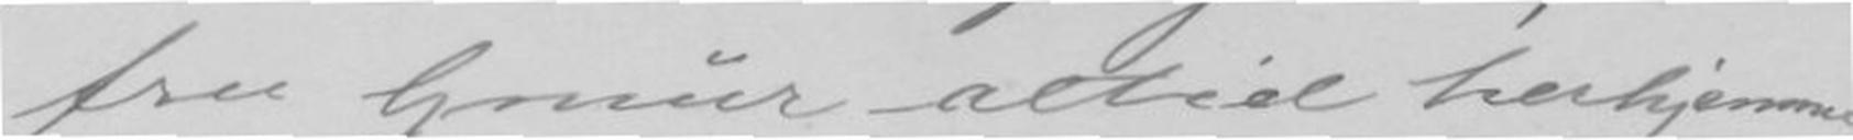fru Gmür - altid her hjemme
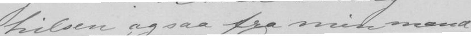hilsen ogsaa fra min mand
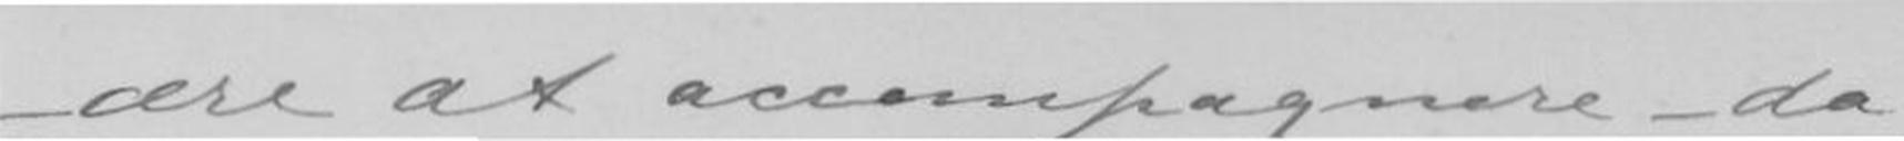- ære at accompagnere, - da
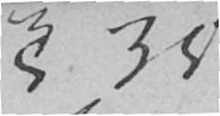§ 35
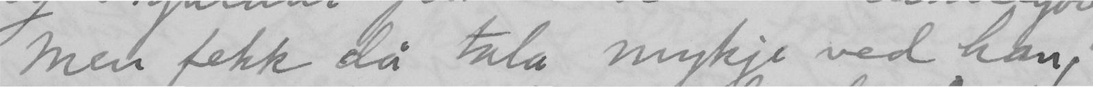Men fekk då tala mykje ved han,
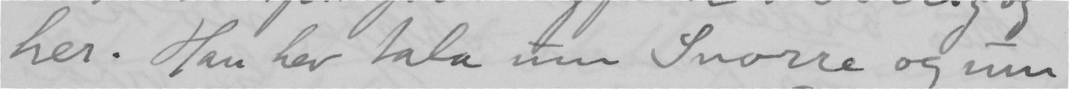her. Han hev tala um Snorre og um
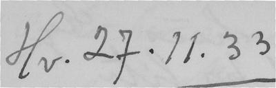Hv. 27.11.33
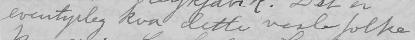eventyrleg kva dette vesle folke
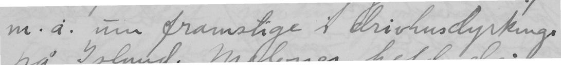m.å. um framstige i drivhusdyrkinga
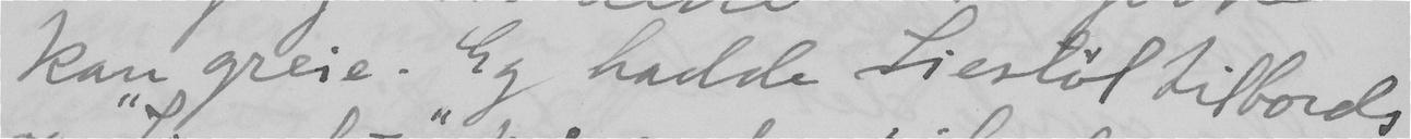kan greie. Eg hadde Liestöl tilbords
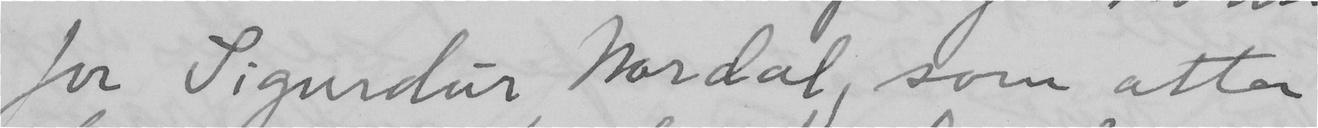for Sigurdur Nordal som atter
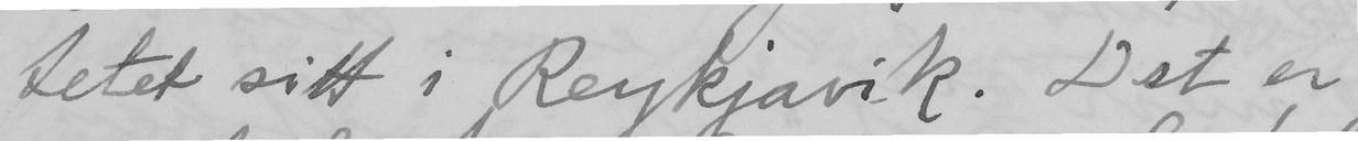tetet sitt i Reykjavik. Det er
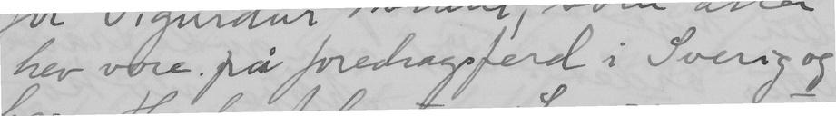hev vore på foredragsferd i Sverig og
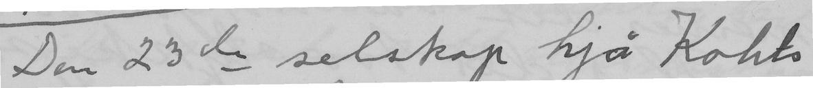Den 23de selskap hjå Kohts
